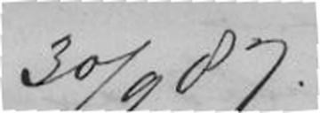30/9.87
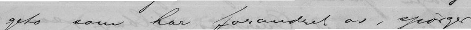geto som har forandret os, spørger
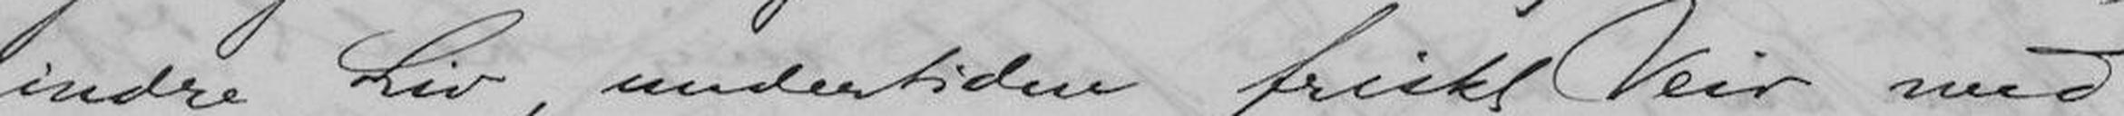indre Liv, undertiden friskt Veir med
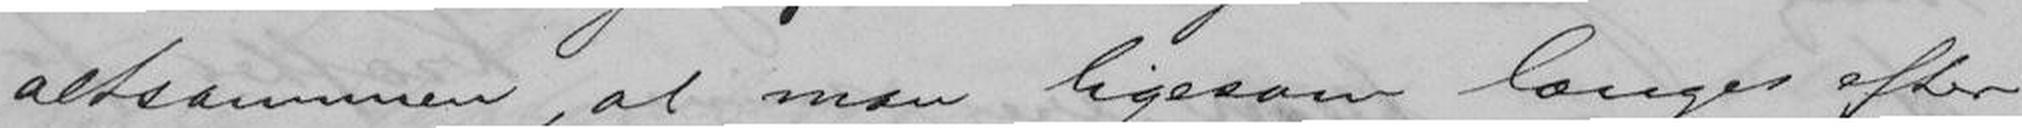altsammen, at man ligesom længes efter
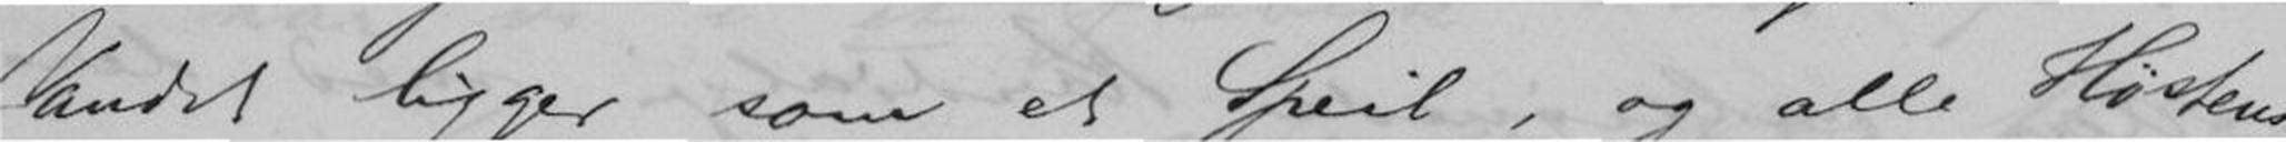Vandet ligger som et Speil, og alle Høstens
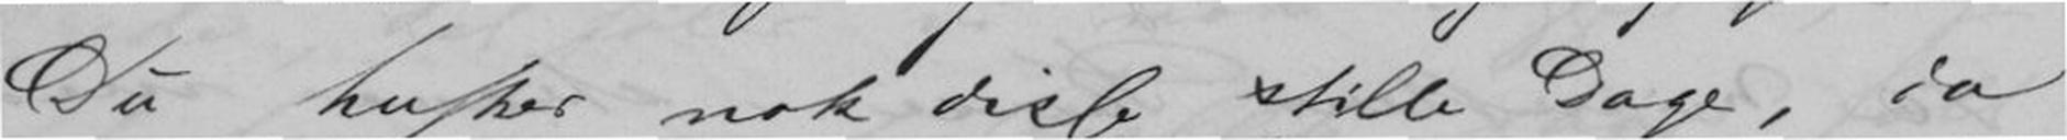Du husker nok disse stille Dage, da
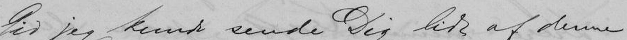Gid jeg kunde sende Dig lidt af denne
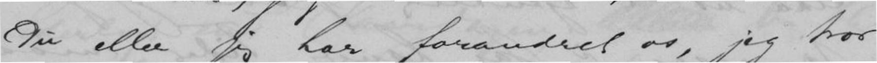Du eller jeg har forandret os, jeg tror
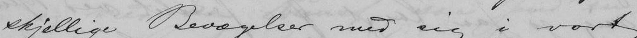skjellige Bevægelser med sig i vort
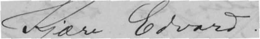Kjære Edvard!
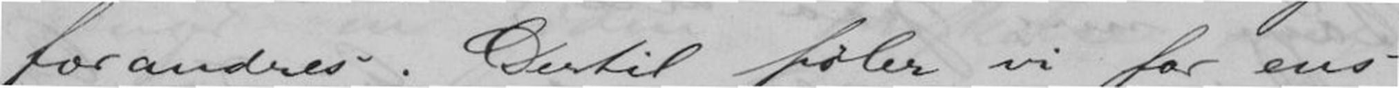forandres. Dertil føler vi for ens
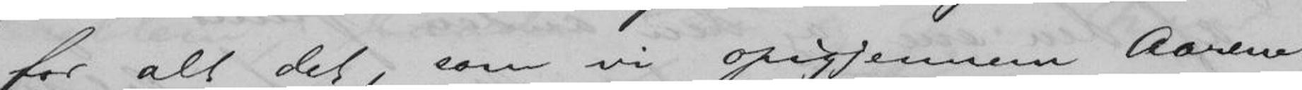for alt det, som vi opigjennem Aarene
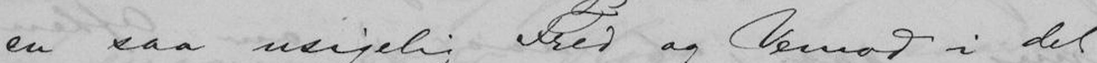en saa usigelig Fred og Vemod i det
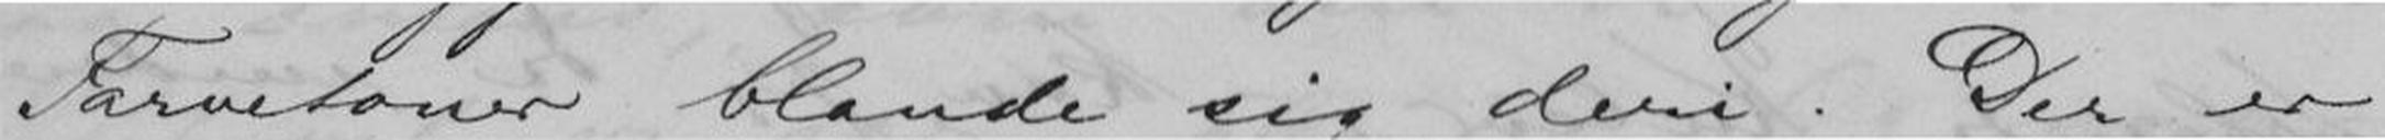Farvetoner blande sig deri. Der er
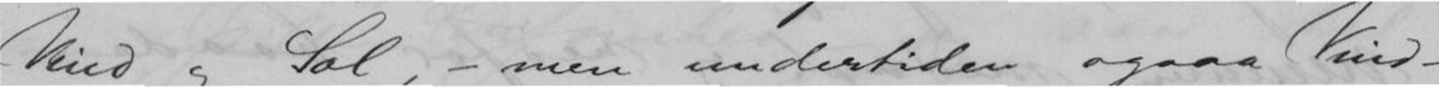Vind og Sol, - men undertiden ogsaa Vind-
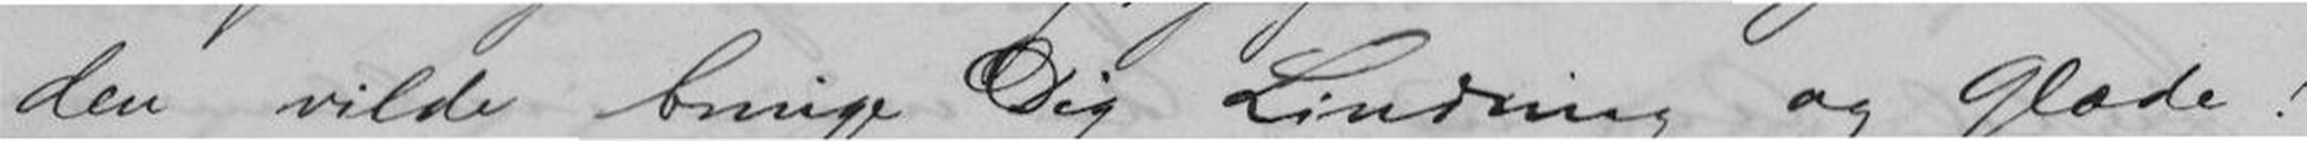den vilde bringe Dig Lindring og Glæde!
